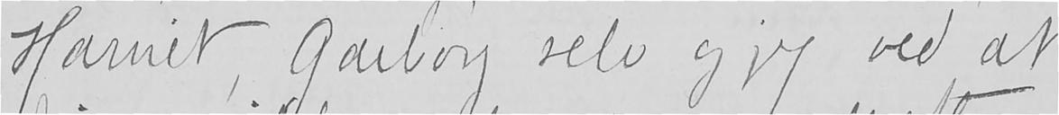Harriet, Garborg selv og jeg ved at
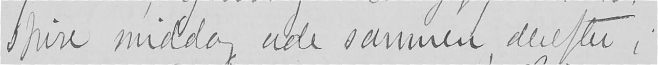spise middag ude sammen, derefter i
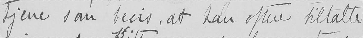tjene som bevis, at han oftere tiltalte
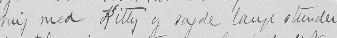mig med Kitty og sagde lange stunder
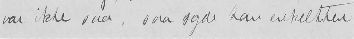var ikke saa, saa sagde han enkelthen
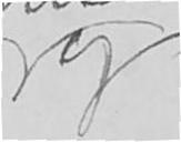og
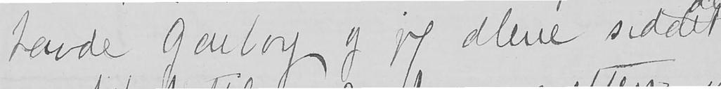havde Garborg og jeg alene siddet
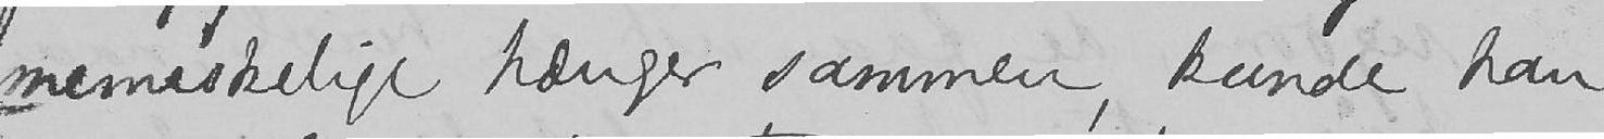menneskelige hænger sammen, kunde han
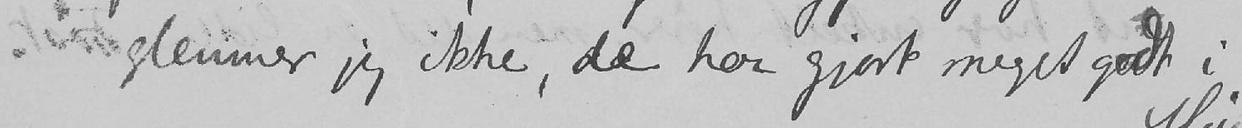glemmer jeg ikke, de har gjort meget godt i
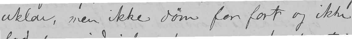uklar, men ikke døm for fort og ikke
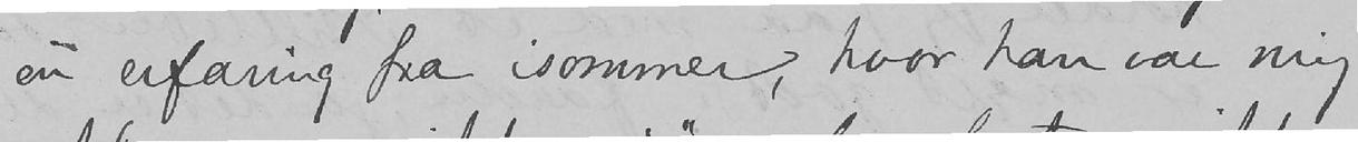en erfaring fra isommer, hvor han var mig
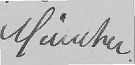München.
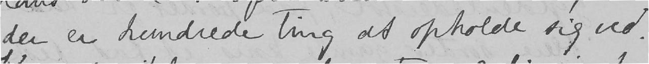der er hundrede ting at opholde sig ved.
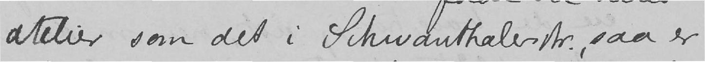atelier som det i Schwanthalerstr., saa er
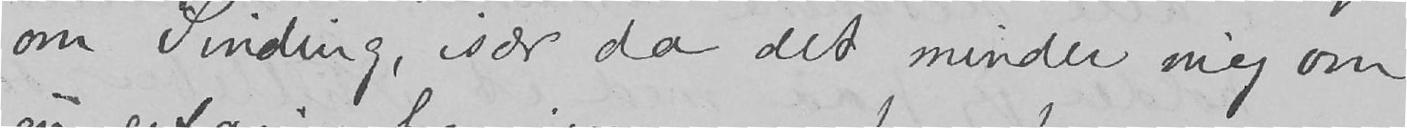om Sinding, især da det minder mig om
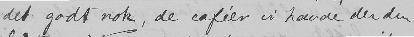det godt nok, de caféer vi havde der den
